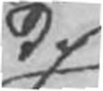de
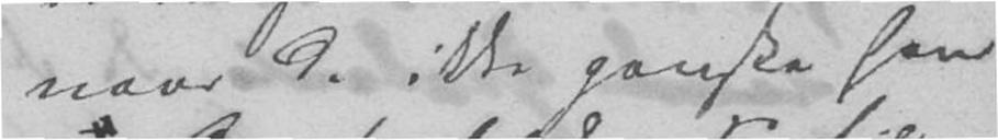naar de ikke ganske have
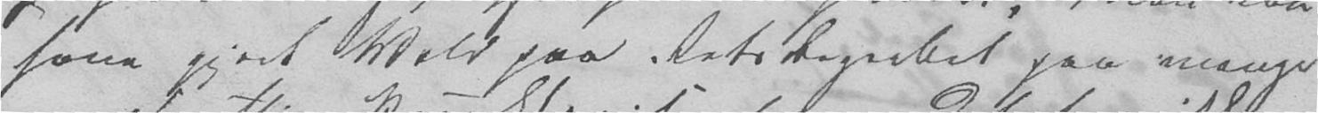have gjort Vold paa Retsbegrebet paa mange
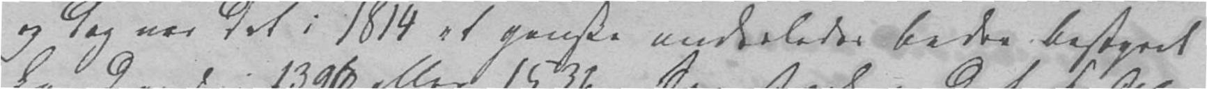og dog var det i 1814 et ganske anderledes bedre bestyret
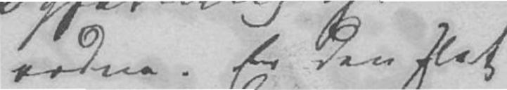ordne. Er den slet
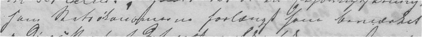som Statsøkonomerne forlængst have bemærket
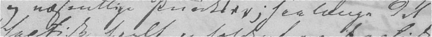og væsentlige Punkter; saa længe det
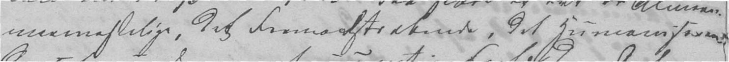menneskelige, det Fremadstræbende, det humaniseren-
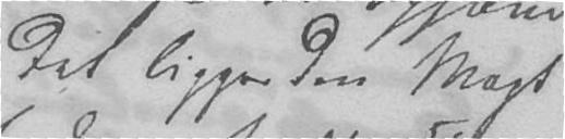Det ligger den Magt
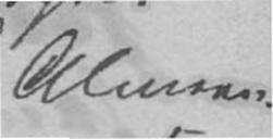Almeen-
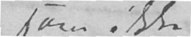som ikke
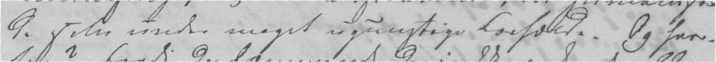de selv under meget ugunstige Forholde. Og hvor-
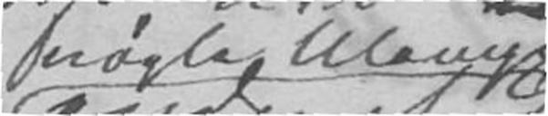nogle Ulemper
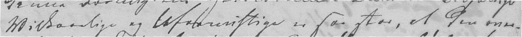Vilkaarlige og Ufornuftige er saa stor, at den over-
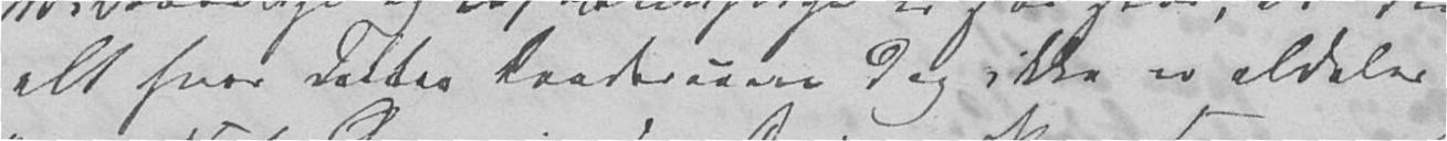alt hvor dettes baadermere dog ikke er aldeles
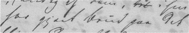har gjort Brud paa det
